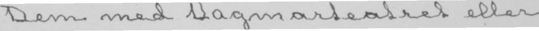Dem med Dagmarteatret eller
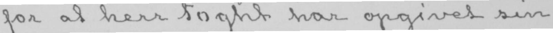for at herr Foght har opgivet sin
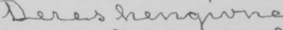Deres hengivne
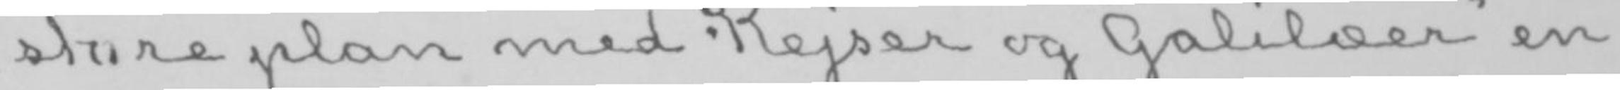store plan med «Kejser og Galilæer» en
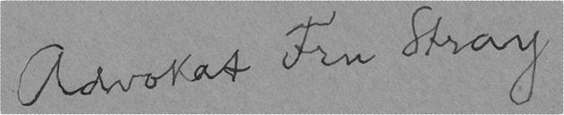Advokat Fru Stray
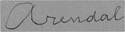Arendal
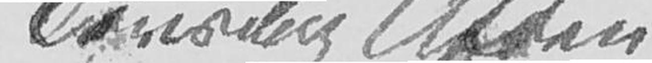Tirsdag Aften
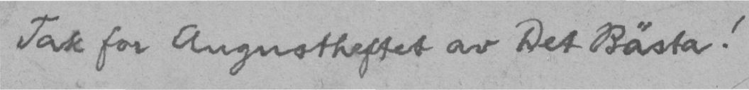Tak for Augustheftet av Det Bästa!
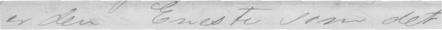er den Eneste som det
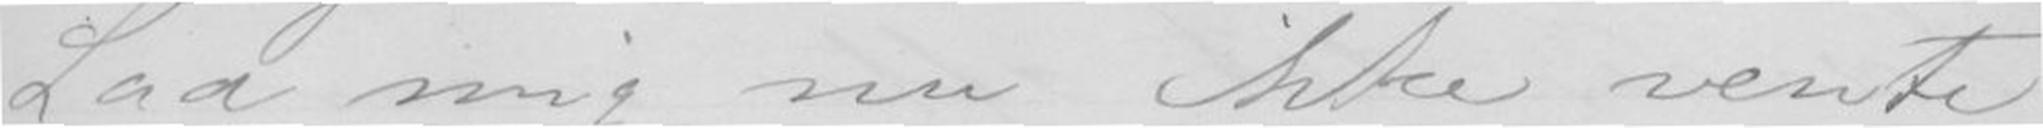Lad mig nu ikke vente
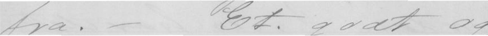fra. - Et godt og
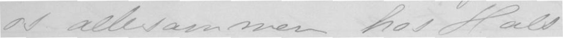os allesammen hos Hals
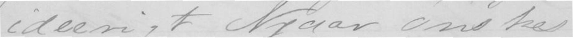ideerigt Nyaar ønskes
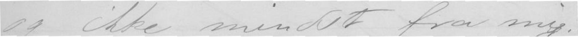og ikke mindst fra mig.
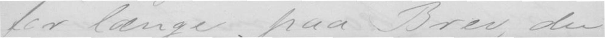for længe paa Brev, du
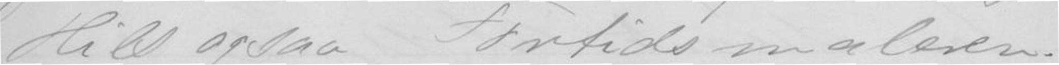Hils ogsaa Fortidsmaleren.
